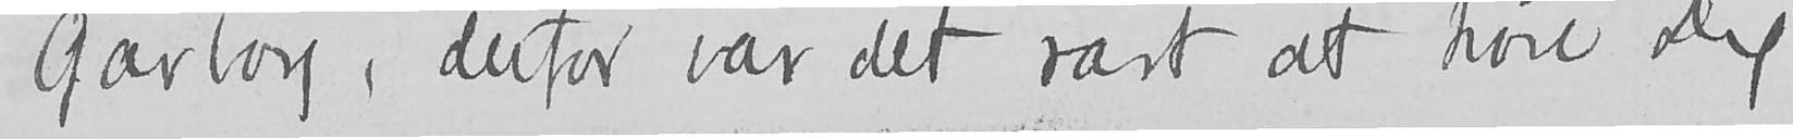Garborg, derfor var det rart at høre Dig
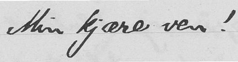Min kjære ven!
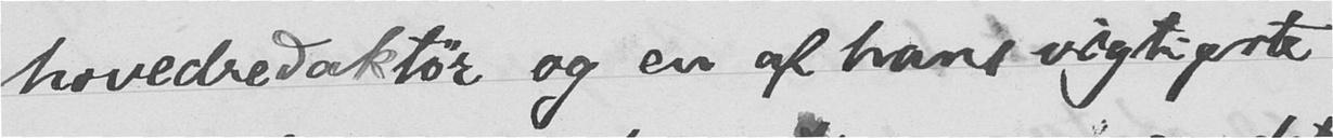hovedredaktør og en af hans vigtigste
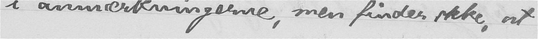i anmærkningerne, men finder ikke, at
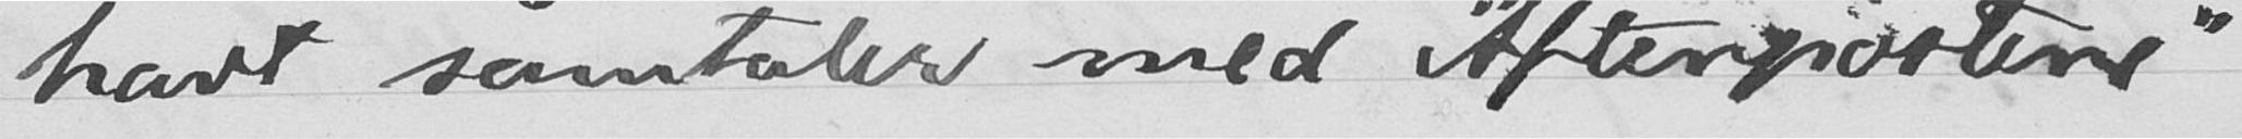havt samtaler med "Aftenpostens"
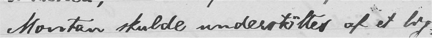Montan skulde understøttes af et lig-
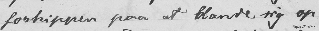fortrippen paa at blande sig op
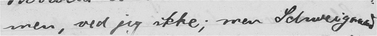men, ved jeg ikke; men Schweigaard
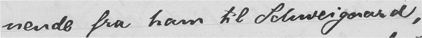nende fra ham til Schweigaard,
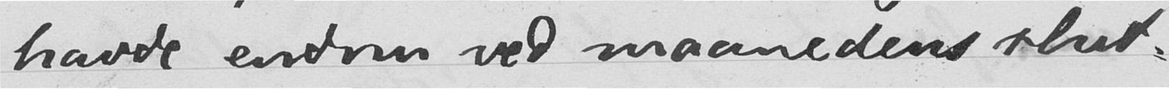havde endnu ved maanedens slut-
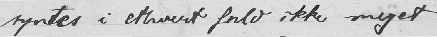syntes i ethvert fald ikke meget
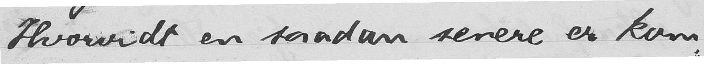Hvorvidt en saadan senere er kom-
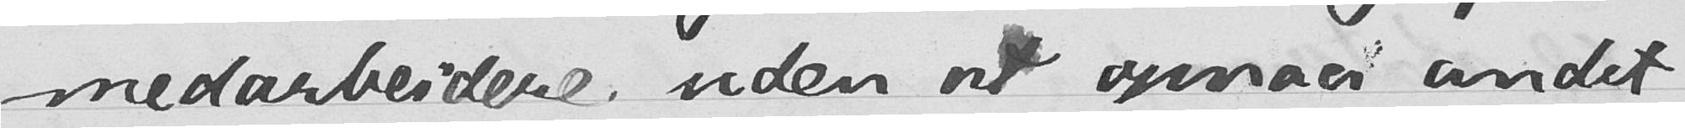medarbeidere, uden at opnaa andet
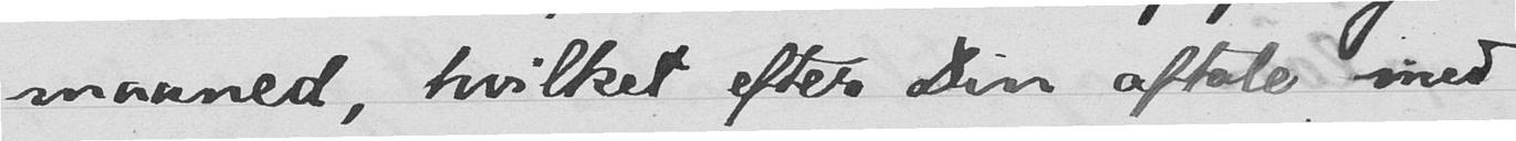maaned, hvilket efter Din aftale med
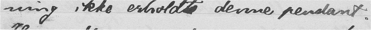ning ikke erholdt denne pendant.
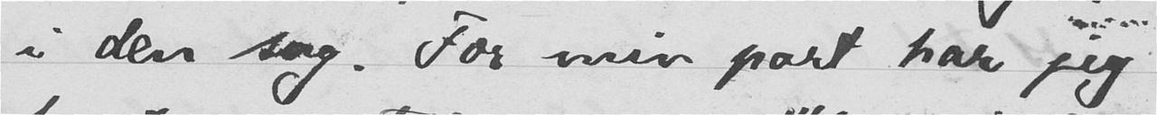i den sag. For min part har jeg
